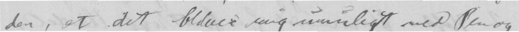den, at det bliver mig umuligt med Pen og
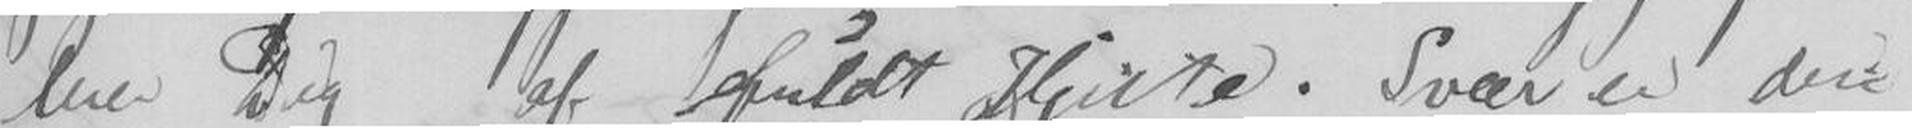lerer Dig af fuldt Hjerte. Svær er den
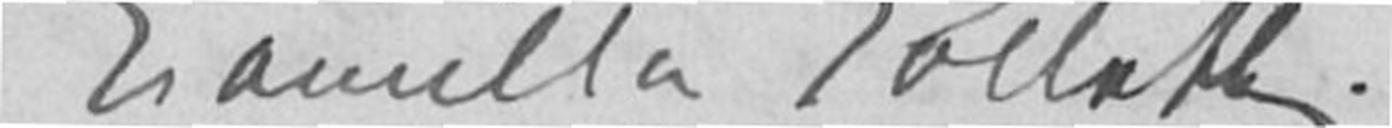Camilla Collett.
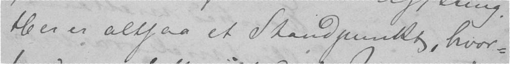Her er altsaa et Standpunkt, hvor-
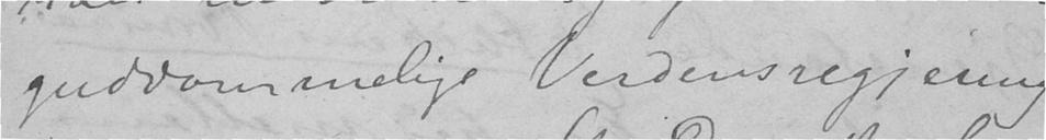guddommelige Verdensregjering.
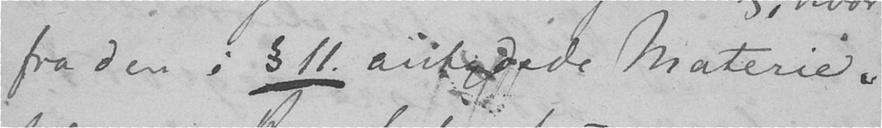fra den i § 11. antydede Materie
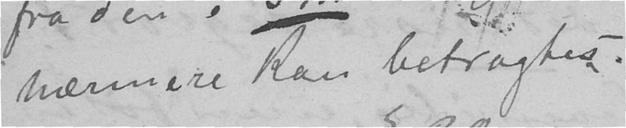nærmere kan betragtes.
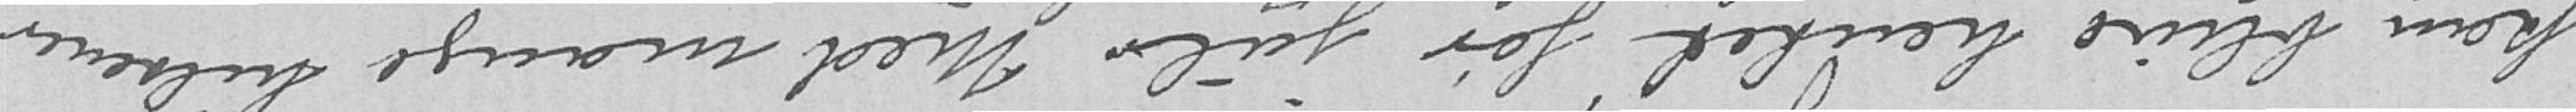kan blive hentet før jul. Med mange hilsener
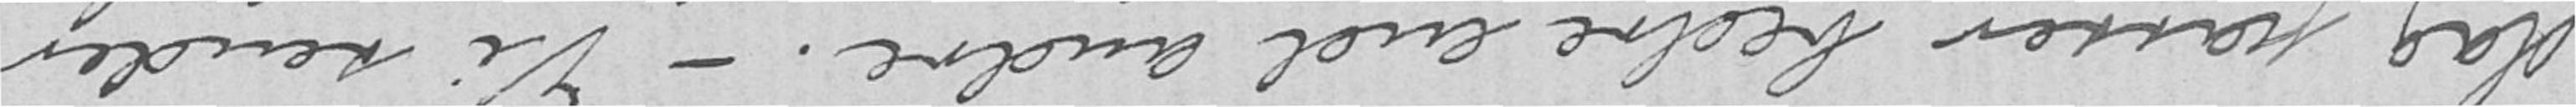dag passer bedre end andre. - Vi sender
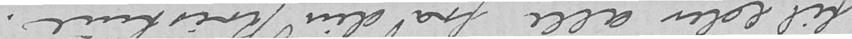til eder alle fra din Kristine.
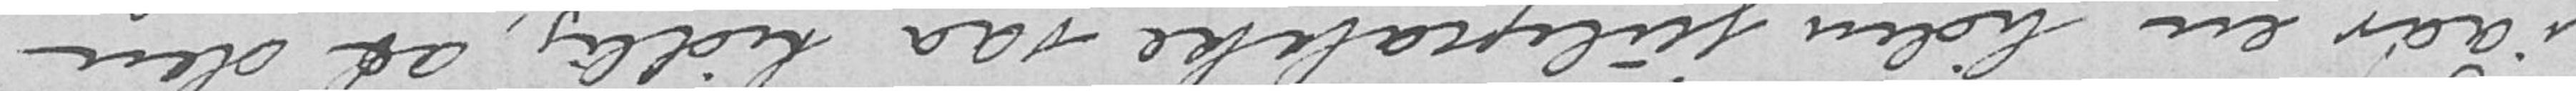i aar en liden julepakke saa tidlig, at den
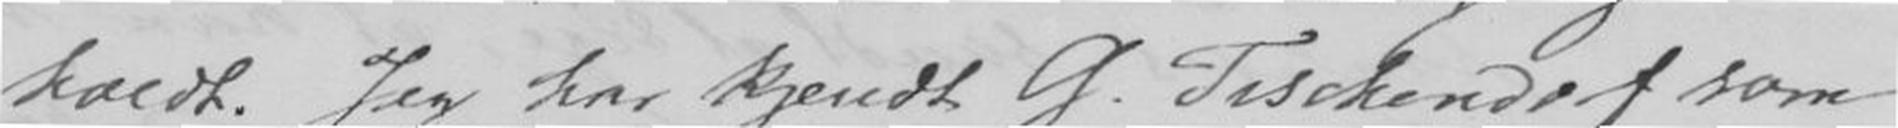holdt. Jeg har kjendt G. Tisckendorf som
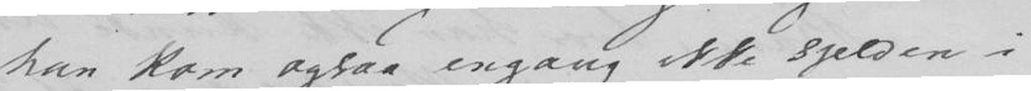han kom ogsaa engang ikke sjelden i
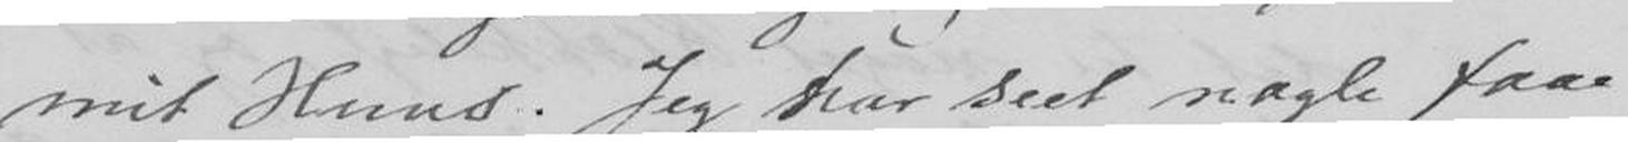mit Huus. Jeg har seet nogle faa
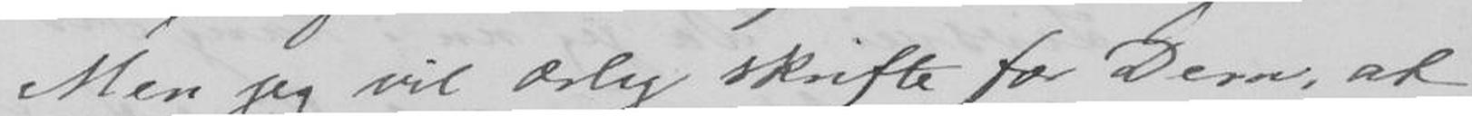Men jeg vil ærlig skrifte for Dem, at
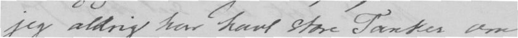jeg aldrig har havt store Tanken om
